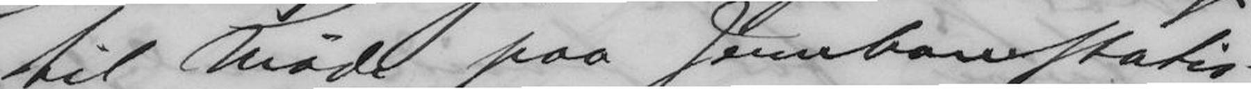til Møde paa Jernbanestatio-
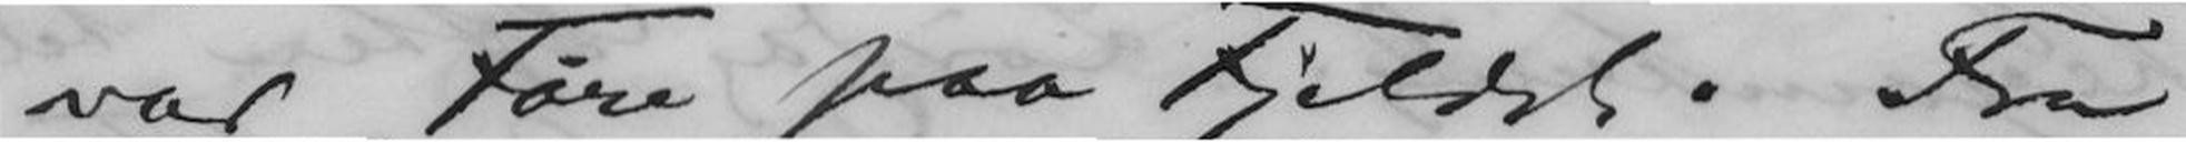var Føre paa Fjeldet. Fra
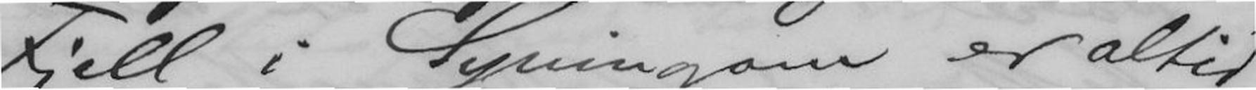Fjell i Syningom er altid
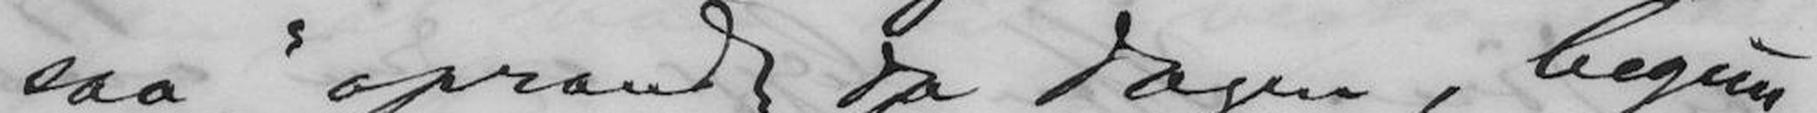saa "oprandt da Dagen, begun-
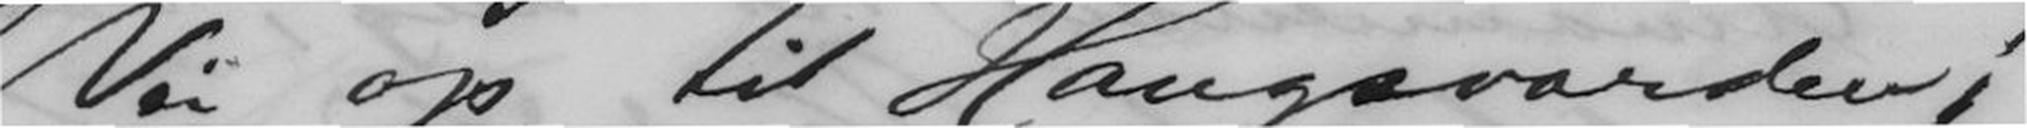Vei op til Haugsvarden;
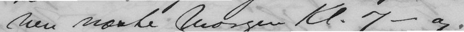nen næste Morgen Kl. 7- og
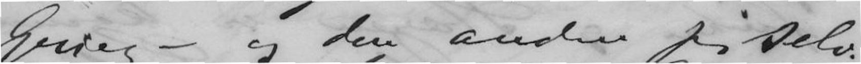Grieg - og den anden jeg selv.
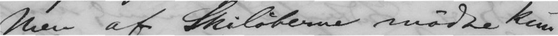Men af Skiløberne mødte kun
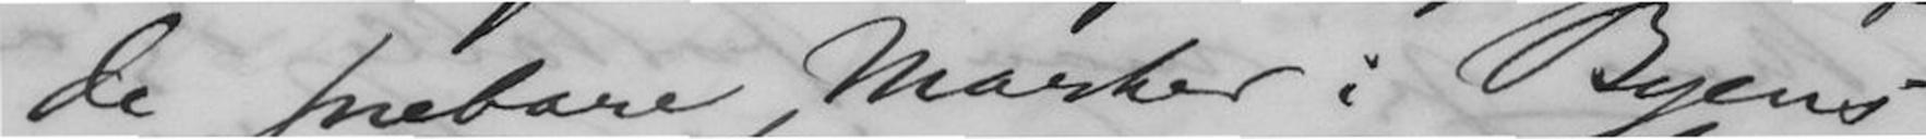de snebare Marker i Byens
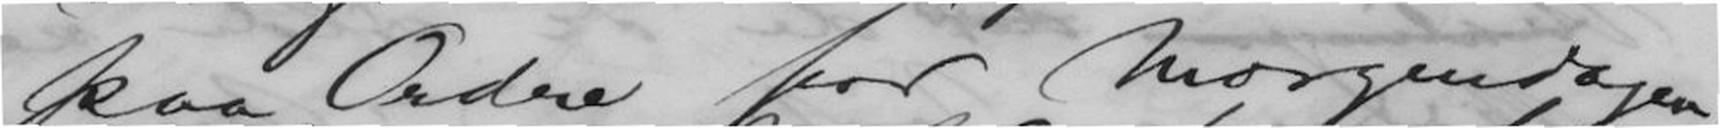aa Ordre for Morgendagen
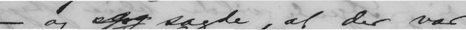- og jeg sagde, at der var
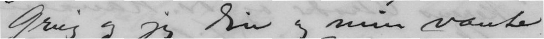Grieg og jeg Din og min vante
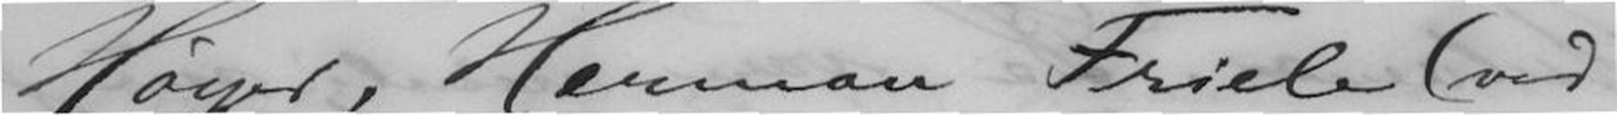Høyer, Herman Friele (ved
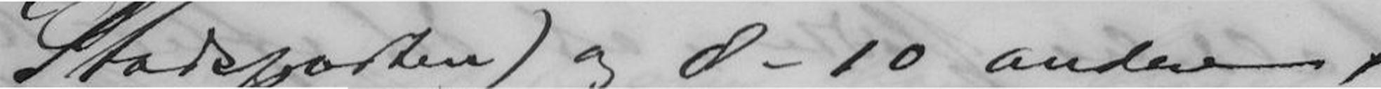Stadsporten) og 8 - 10 andre,
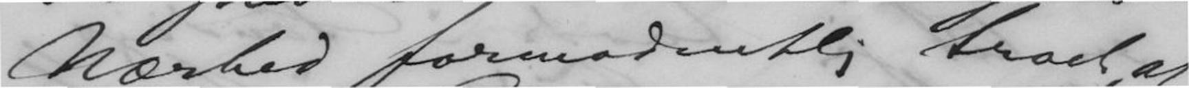Nærhed formodentlig troet, at
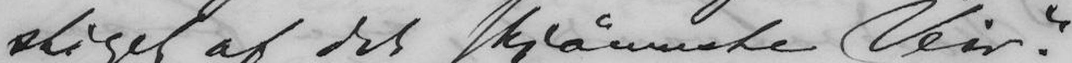stiget af det skønneste Veir".
